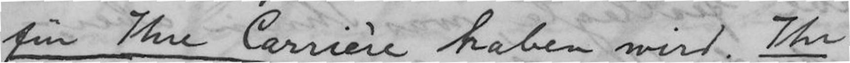für Ihre Carrière haben wird. Ihr
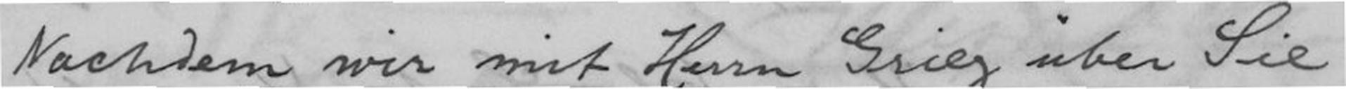Nachdem wir mit Herrn Grieg über Sie
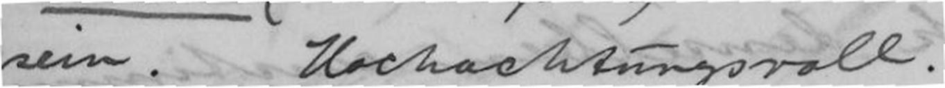sein. Hochachtungsvoll.
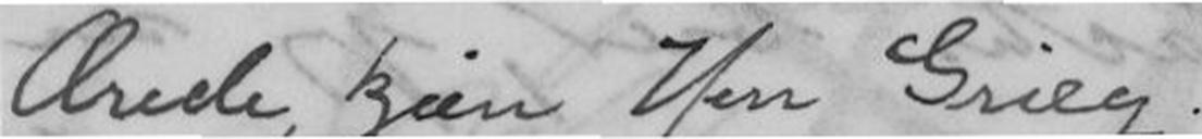Ærede, kjære Herr Grieg!
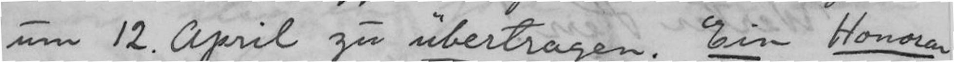um 12.April zu übertragen. Ein Honorar
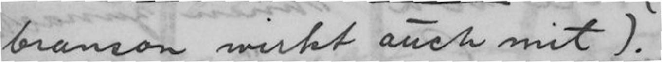branson wirkt auch mit).
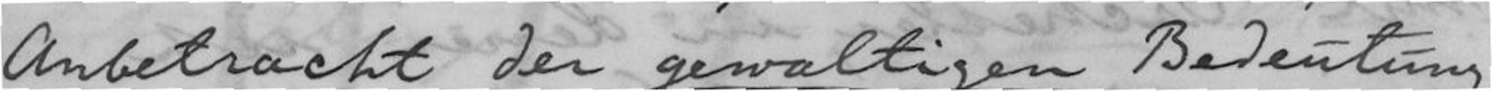Anbetracht der gewaltigen Bedeutung,
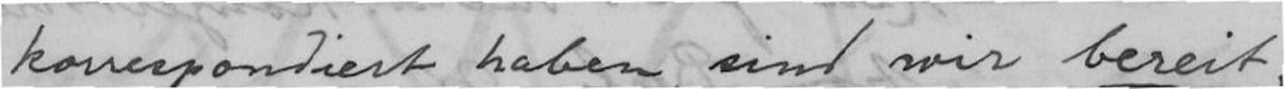korrespondiert haben, sind wir bereit,
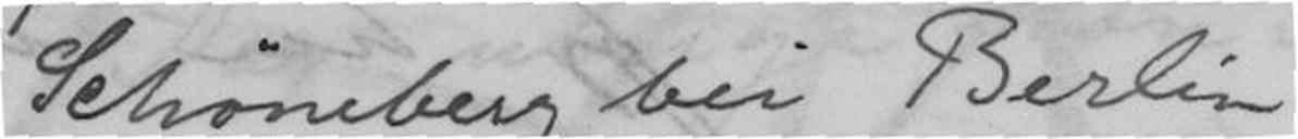Schöneberg bei Berlin
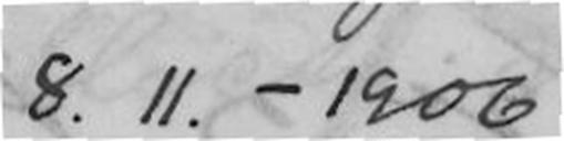8.11.-1906
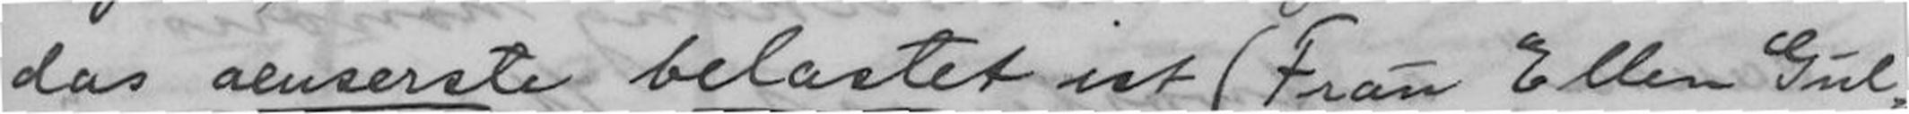das aeuserste belastet ist (Frau Ellen Gul-
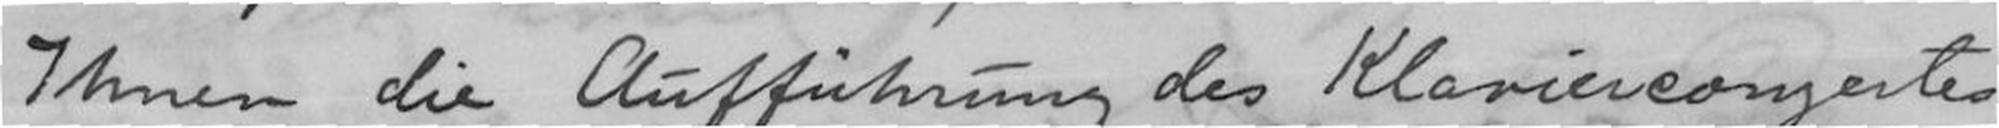Ihnen die Aufführung des Klavierconzertes
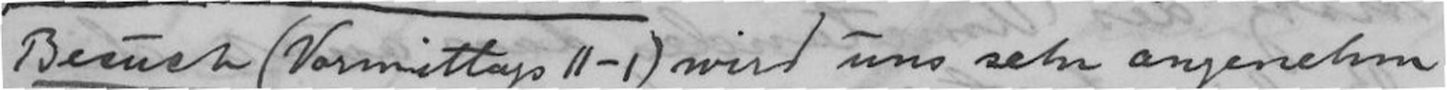Besuch (Vormittags 11-1) wird uns sehr angenehm
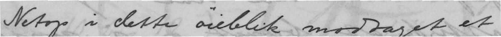Netop i dette öieblik modtaget et
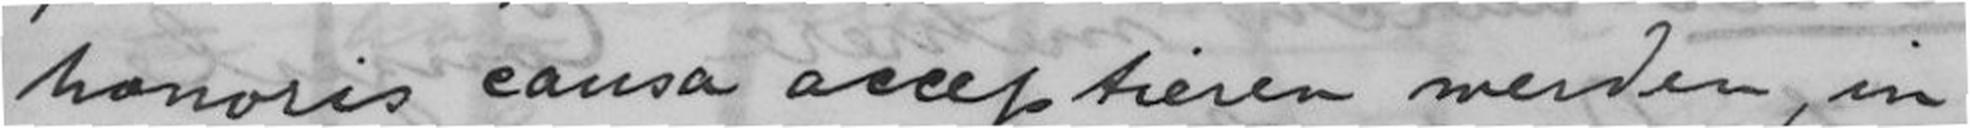honoris causa acceptieren werden, in
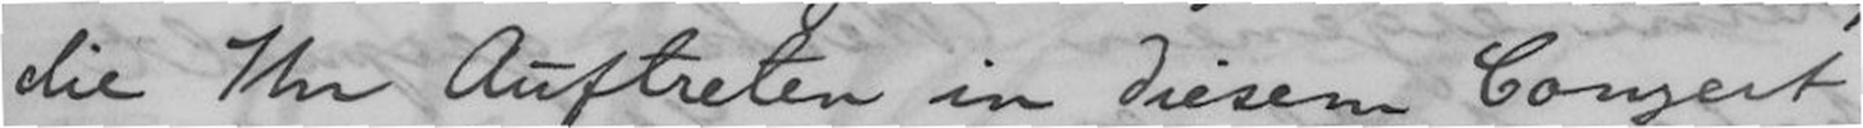die Ihr Auftreten in diesem Conzert
